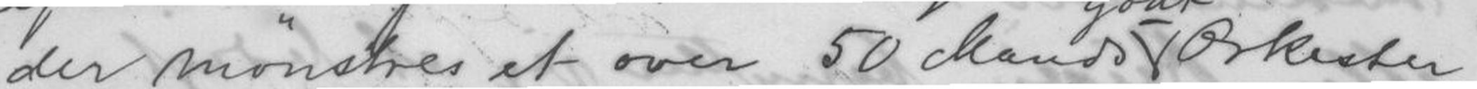der mønstres et over 50 Mands Orkester,
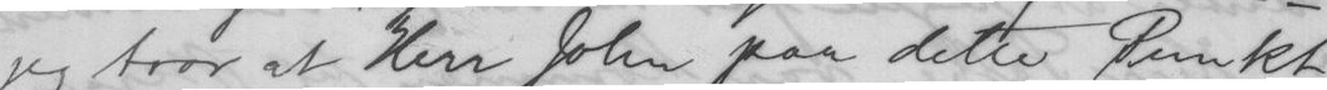jeg tror at Herr John paa dette Punkt
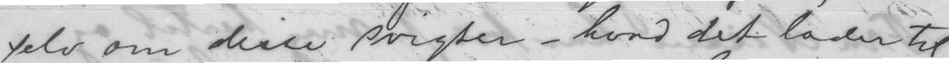selv om disse svigter - hvad det lader til
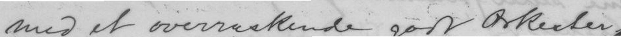med et overraskende godt Orkester.
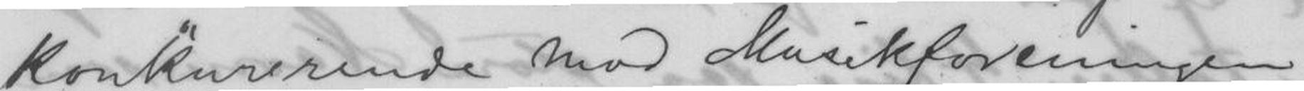konkurerende mod Musikforeningen
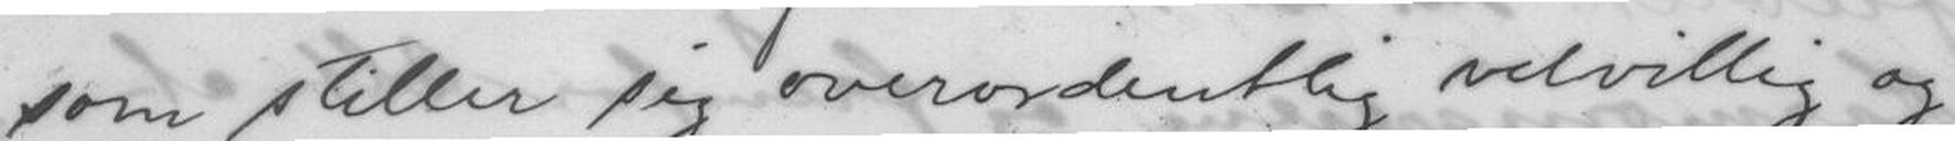som stiller sig overordentlig velvillig og
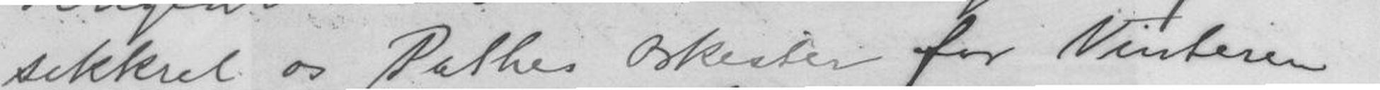sikkret os Pathes Orkester for Vinteren
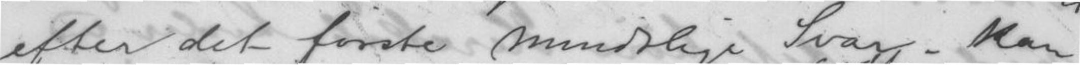efter det første mundtlige Svar - kan
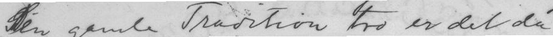Den gamle Tradition tro er det da
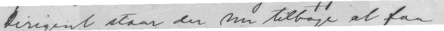Dirigent staar der nu tilbage at faa
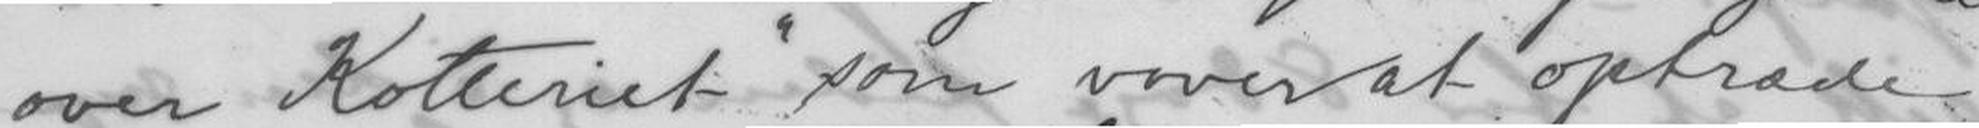over Kotteriet som vover at optræde
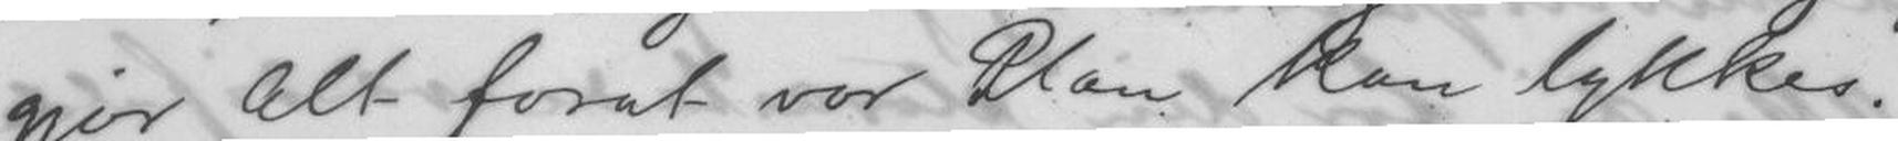gjør Alt forat vor Plan kan lykkes.
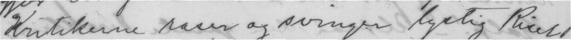Kritikerne raser og svinger lystig Riset
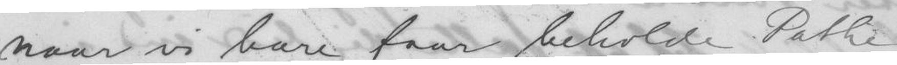naar vi bare faar beholde Pathe
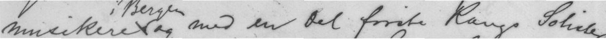musikere og med en Del første Rangs Solister
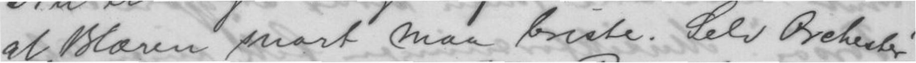at Blæren snart maa briste. Selv Orchester
